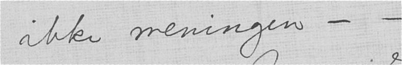ikke meningen - -
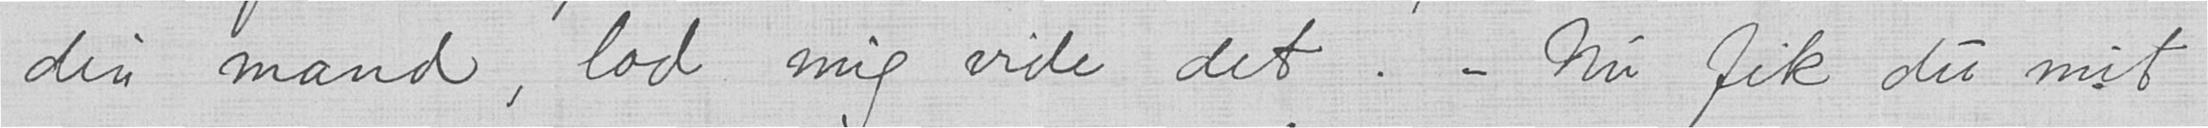din mand, lod mig vide det. - Nu fik du mit
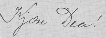Kjære Dea!
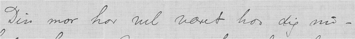Din mor har vel været hos dig nu -
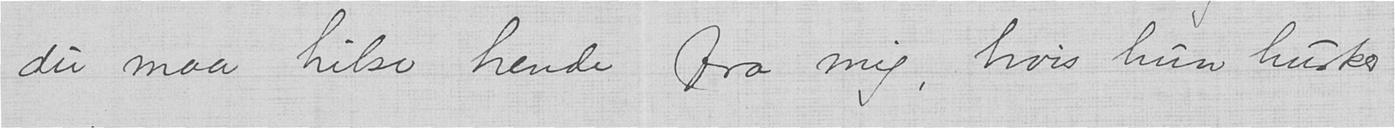du maa hilse hende fra mig, hvis hun husker
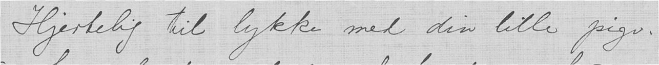Hjertelig til lykke med din lille pige.
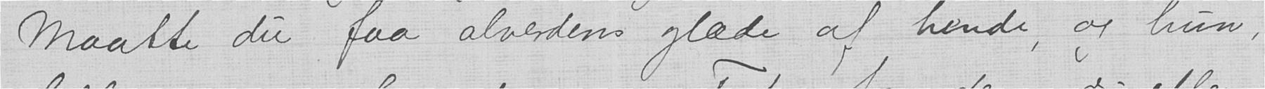Maatte du faa alverdens glæde af hende, og hun,
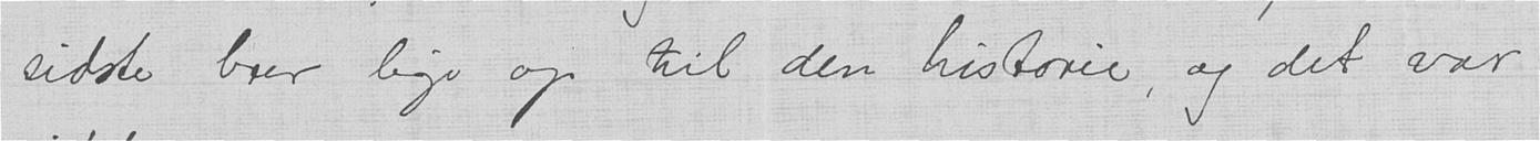sidste brev lige op til den historie, og det var
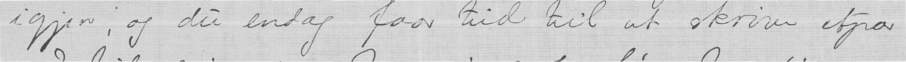igjen, og du endog faar tid til at skrive etpar
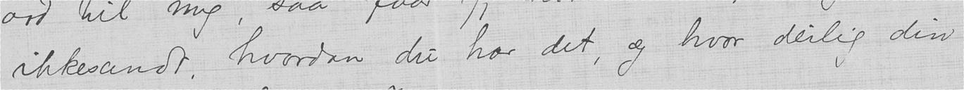ikkesandt, hvordan du har det, og hvor deilig din
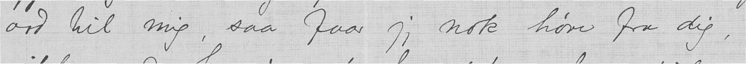ord til mig, saa faar jeg nok høre fra dig,
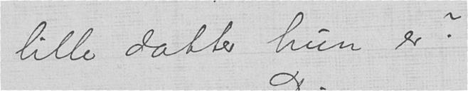lille datter hun er?
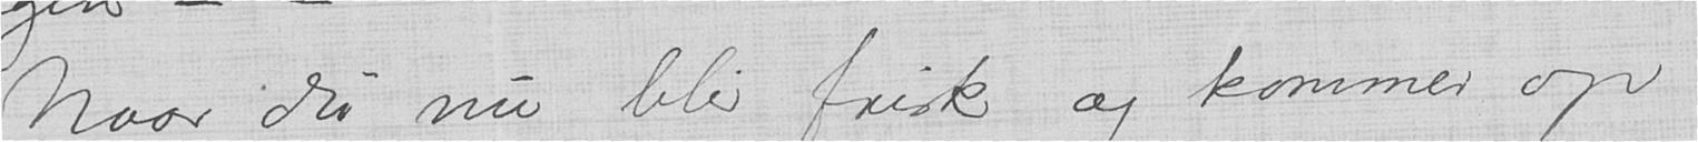Naar du nu blir frisk og kommer op
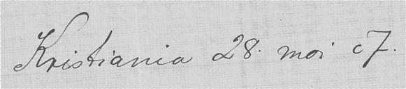Kristiania 27. mai 07.
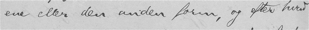ene eller den anden form, og efter hvad
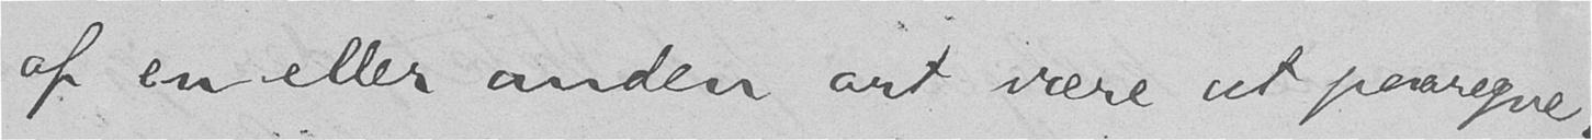af en eller anden art være at paaregne.
